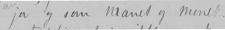ja og som Manet og Monet.
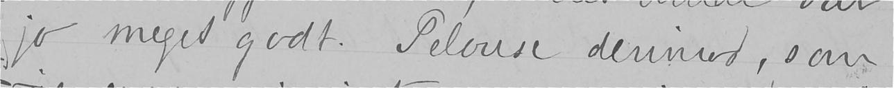jo meget godt. Pelouse derimod, som
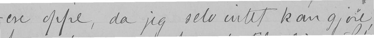ere oppe, da jeg selv intet kan gjøre,
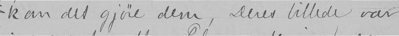kan det gjøre dem, Deres billede var
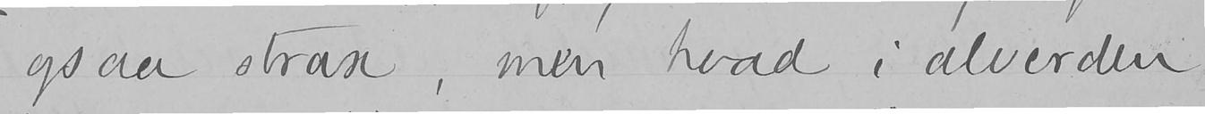ogsaa strax, men hvad i alverden
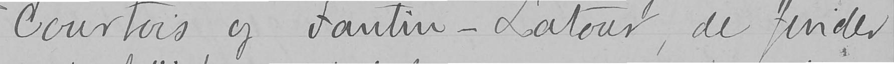Courtois og Fantin-Latour, de finder
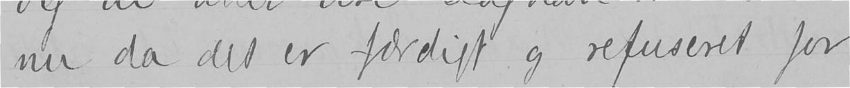nu da det er færdigt og refuseret for
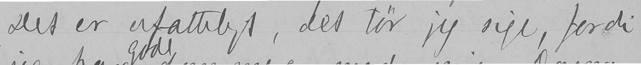Det er ufatteligt, det tør jeg sige, fordi
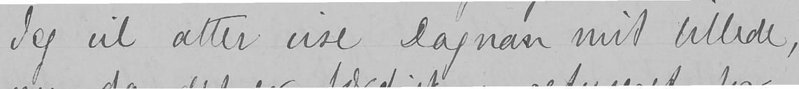Jeg vil atter vise Dagnan mit billede,
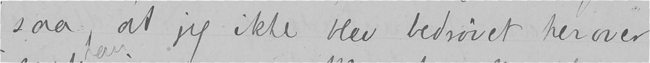saa at jeg ikke blev bedrøvet herover
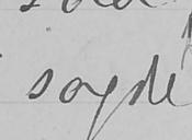sagde
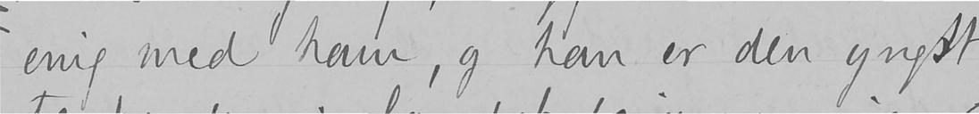enig med ham, og han er den yngst
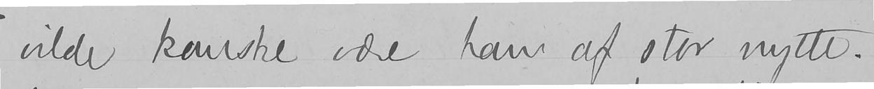vilde kanske være ham af stor nytte.
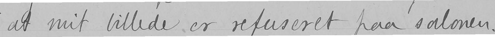at mit billede er refuseret paa salonen.
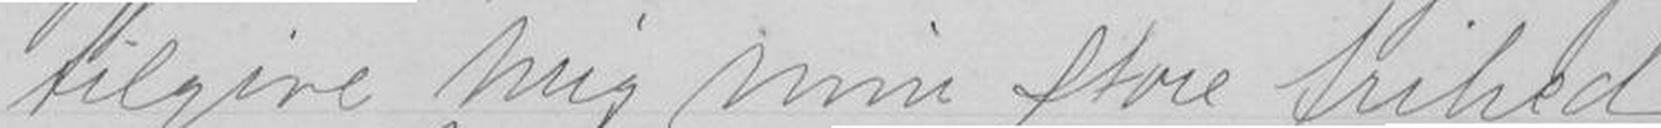tilgive mig min store frihed
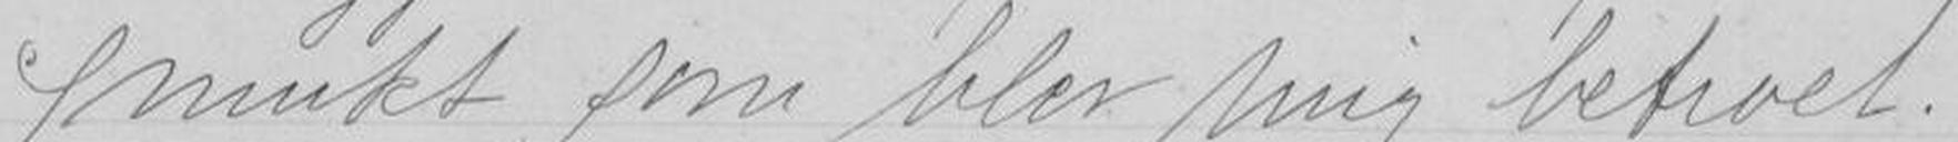smukt, som blev mig betroet
